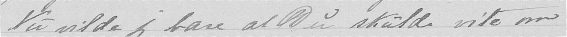Nu vilde jeg bare at Du skulde vite om
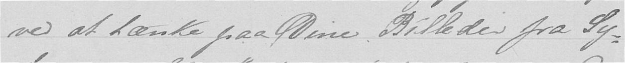ved at tænke paa Dine Billeder fra Sy-
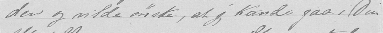den og vilde ønske, at jeg kunde gaa i Din
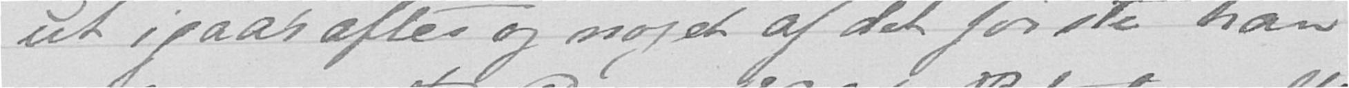ut igaaraftes og noget af det første han
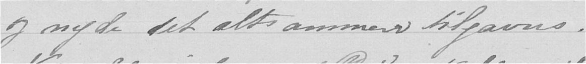og nyde det altsammen tilgavns.
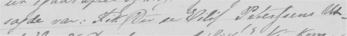sagde var: Fik Du se Eilif Peterssens Ut-
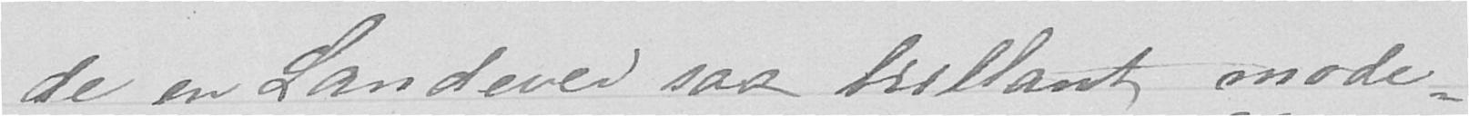de en Landevei saa brillant mode-
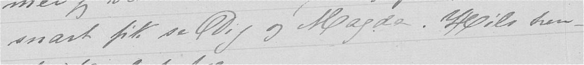snart fik se Dig og Magda. Hils hen-
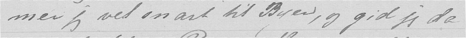mer jeg vel snart til Byen, og gid jeg da
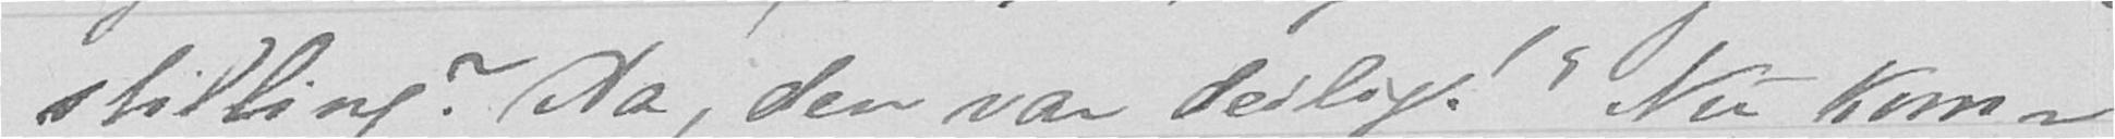stilling? Aa, den var deilig! Nu kom-
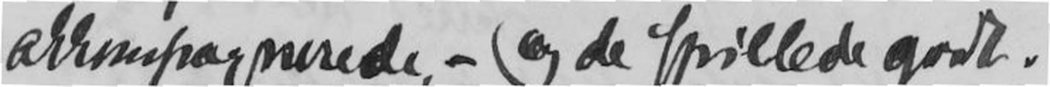akkompagnerede, - og de spillede godt.
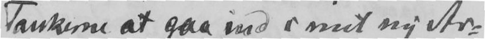Tankerne at gaa ind i mit ny Ar-
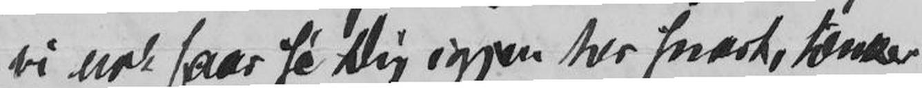vi nok far se Dig igjen her snart, tænker
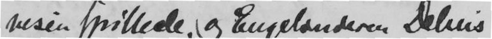nesen spillede, og Engelænderen Delius
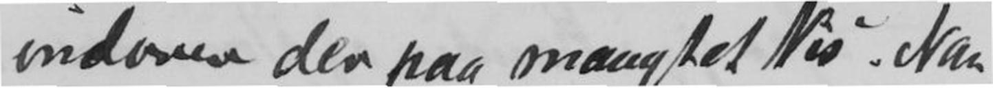indover der paa mangtet Vis. Naa
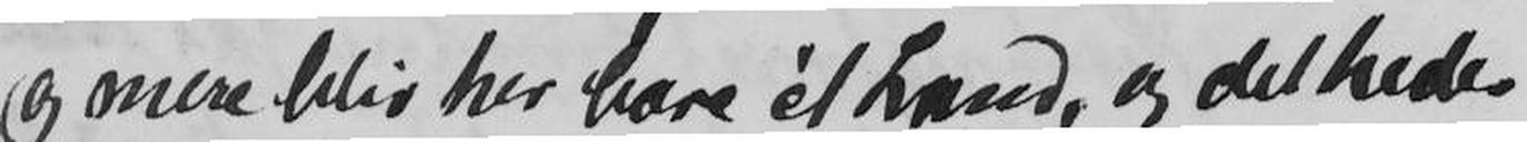og mere blir her bare et Land, og det heder
